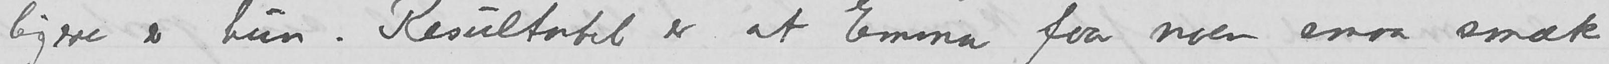ligere er hun. Resultatet er at Emma faar noen smaa smæk
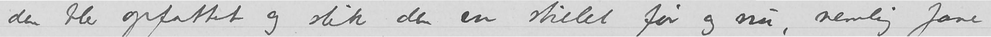den ble opfattet og slik den er stillet før og nu, nemlig Jane
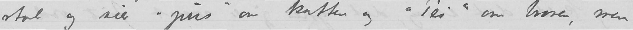stol og sier «pus» om katten og «Pei» om broren, men
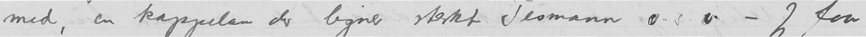med, en kappelan der ligner stærkt Tesmann o.s.v.  jeg faar
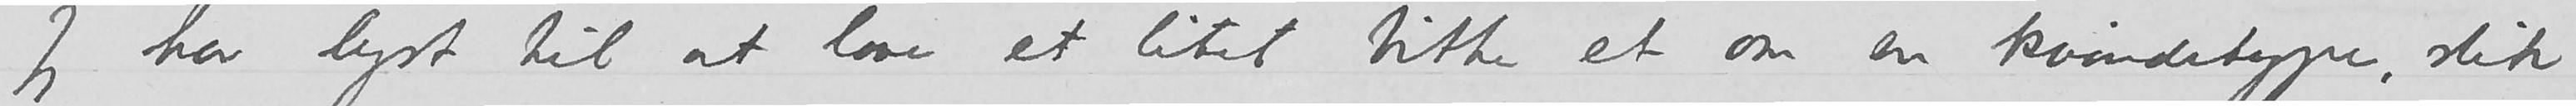Jeg har lyst til at lave et litet bitte et om en kvindetype, slik
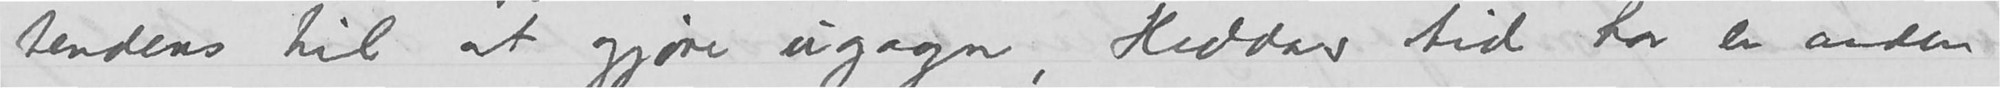tendens til at gjøre ugagn, Heddas tid har en anden
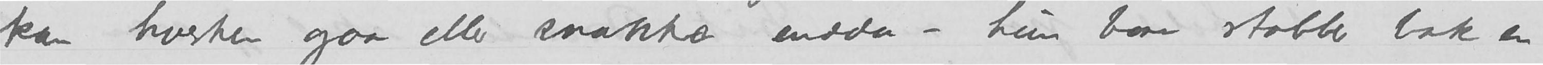kan hverken gaa eller snakke endda -hun bare stabber bak en
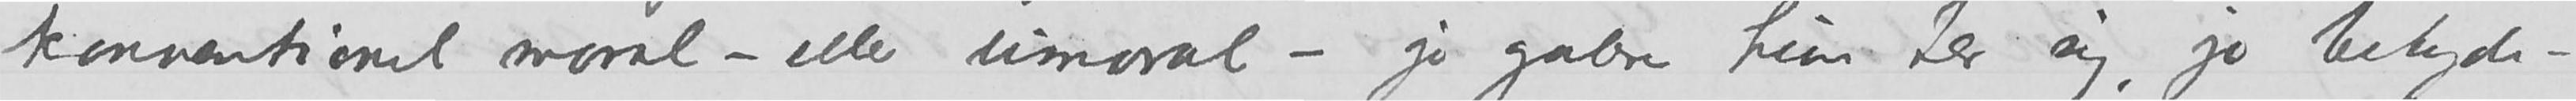konventionel moral - eller umoral -jo galere hun ter sig, jo betyde
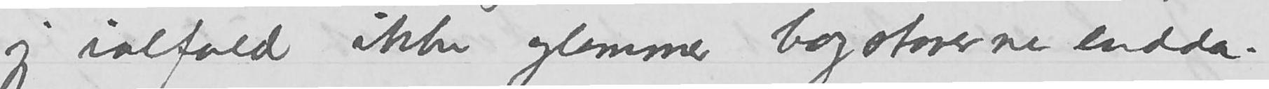jeg ialfald ikke glemmer bogstaverne endda.
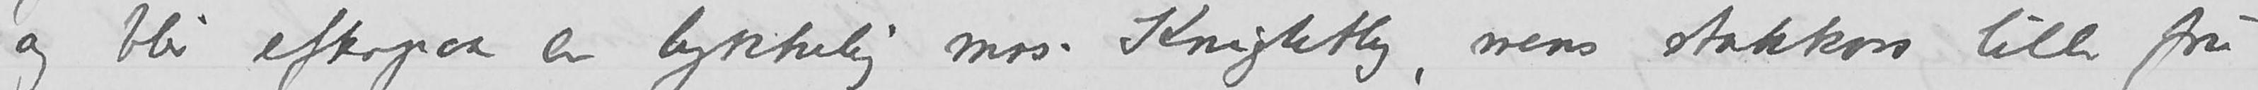og blir efterpaa en lykkelig Mrs. Knightly, mens stakkars lille fru
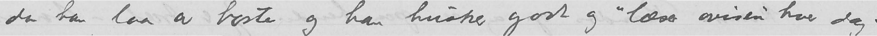da han laa av hoste og han husker godt og «læser avisen» hver dag
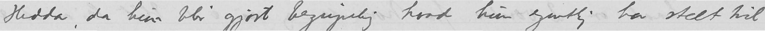Hedda, da hun blir gjort begripelig hvad hun egentlig har stelt til
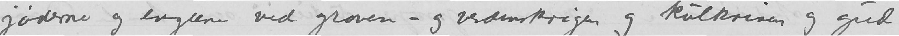jøderne og englene ved graven- og verdenskrigen og kulkrisen og gud
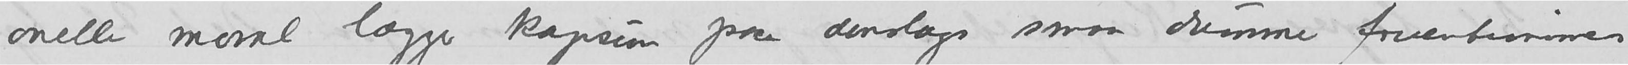nelle moral lægger kapsuner paa den slags smaa dumme fruentimmers
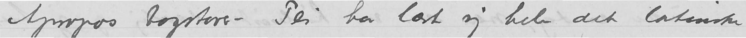Apropos bogstaver-Pei har lært sig hele det latinske
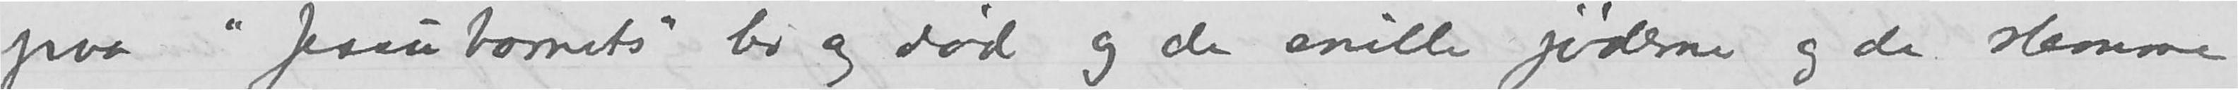paa «Jessu barnets» liv og død og de snille jøderne og de slemme
